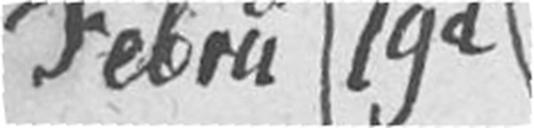Febru 19d
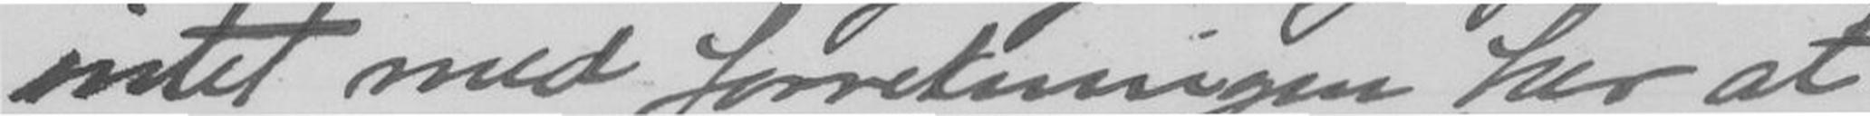intet med forretningen her at
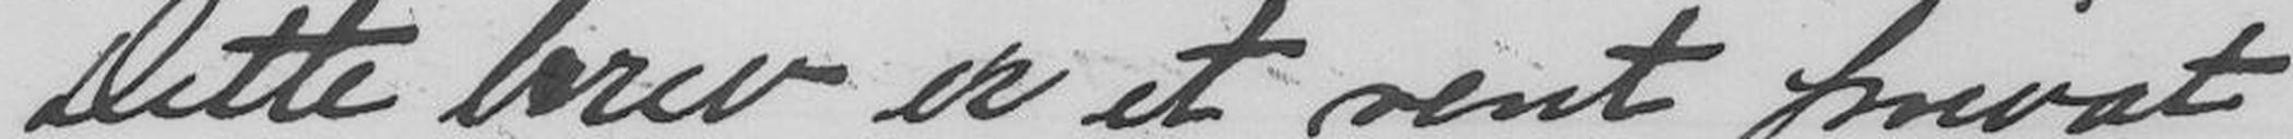Dette brev er et rent privat
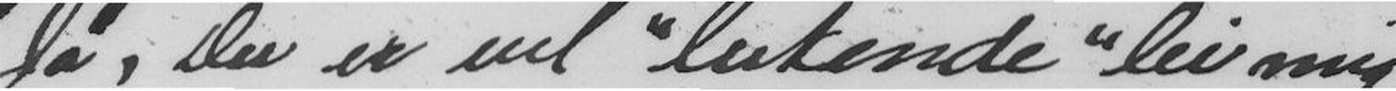Ja, Du er vel "lutende" lei mig
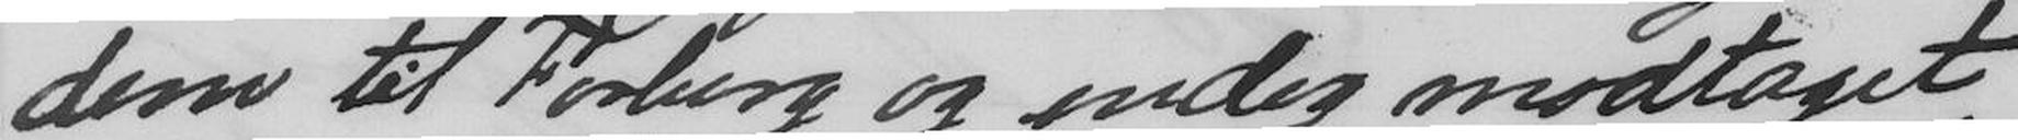dem til Forberg og endog modtaget
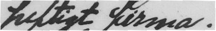heftigt firma.
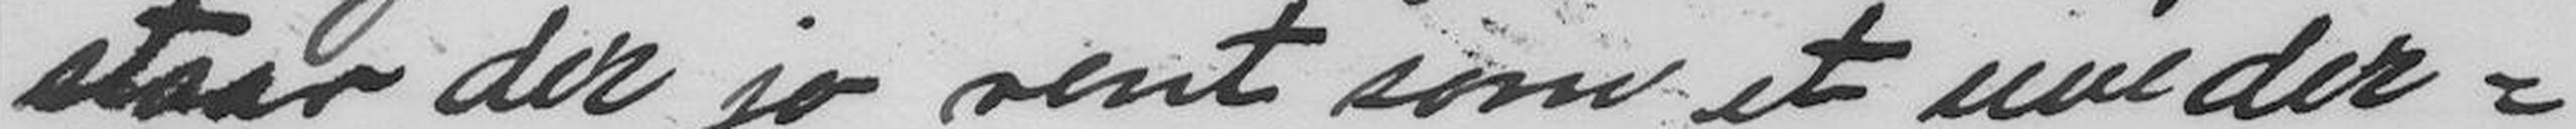staar der jo rent som et uveder-
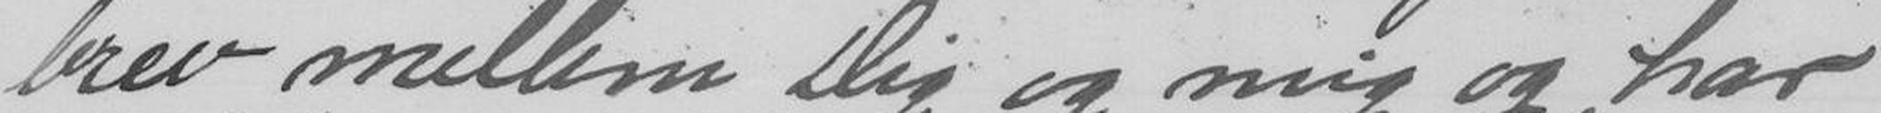brev mellem Dig og mig og har
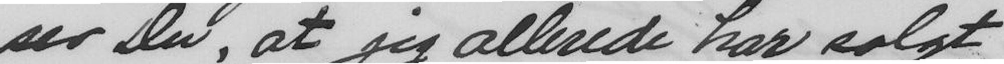ser Du, at jeg allerede har solgt
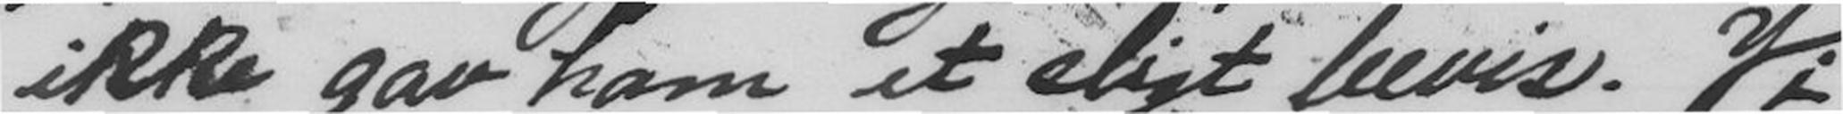ikke gav ham et sligt bevis. Vi
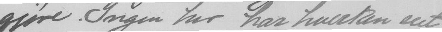gjøre. Ingen her har hverken seet
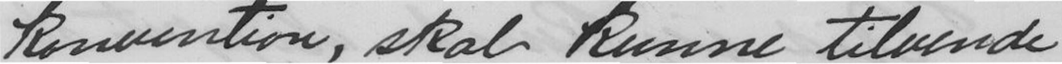konvention, skal kunne tilvende
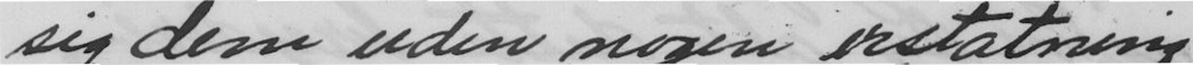sig dem uden nogen erstatning
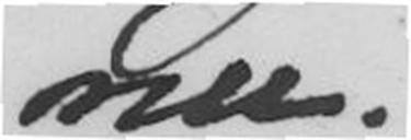nu.
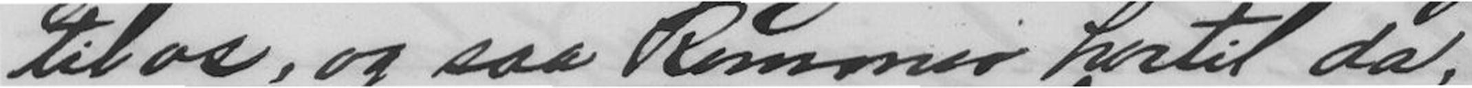til os, og saa kommer hertil da,
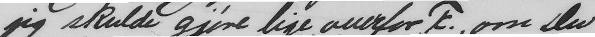jeg skulde gjøre lige overfor F., om Du
